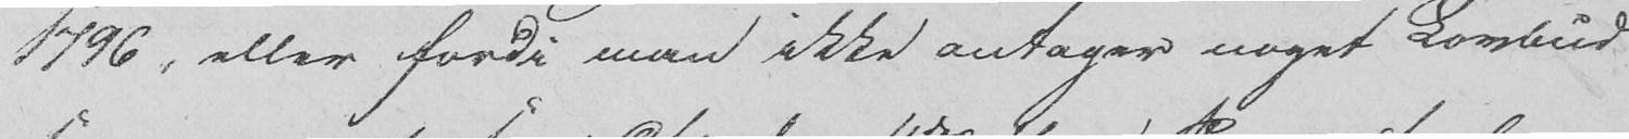1796, eller fordi man ikke antager noget Lovbud
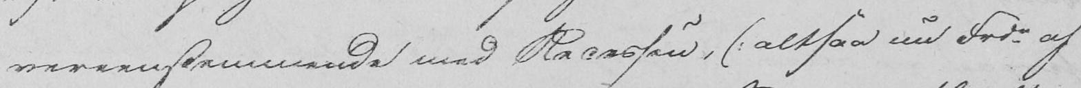vereenstemmende med Recessen, (: altsaa nu Frdn af
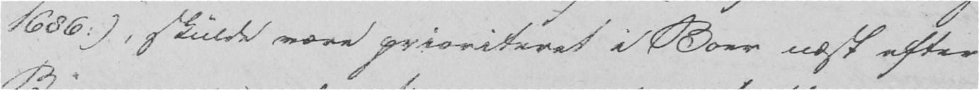1686 :), skulde være prioriteret i Boer næst efter
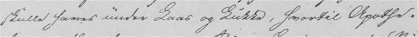skulle haves under Laas og Lukke, hvortil Apothe-
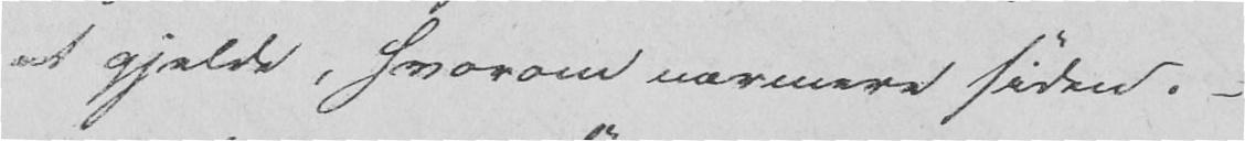at gjelde, hvorom nærmere siden. -
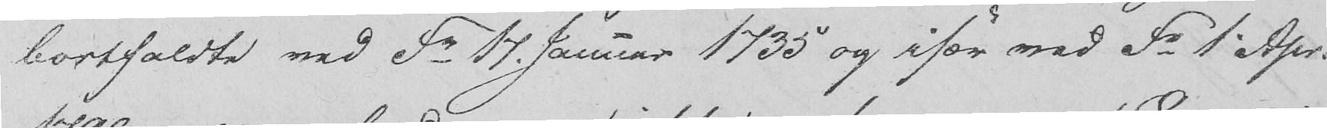bortfaldte ved Fr 17. Januar 1735 og især ved Fr 1. Apr.
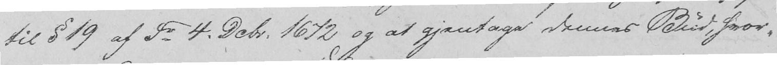til § 19 af Fr 4. Dcbr. 1672 og at gjentage dennes Bud, hvor-
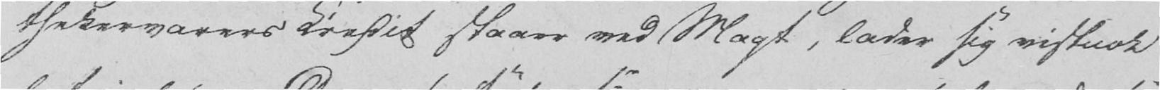thekervarers Credit staaer ved Magt, lader sig vistnok
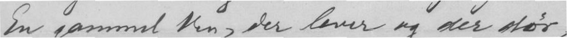En gammel Ven, der lever og der dør,
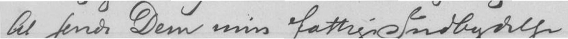at sende Dem min fattige Indbydelse
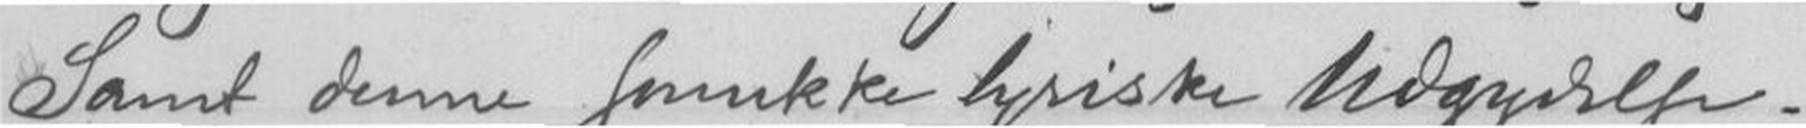Samt denne smukke lyriske Udgydelse.
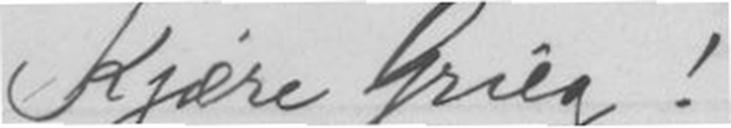Kjære Grieg!
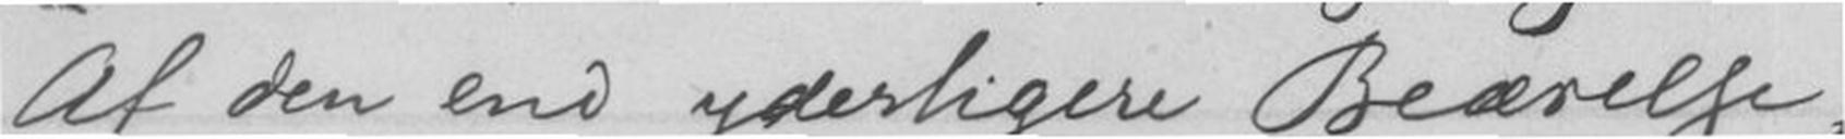Af den end yderligere Beærelse,
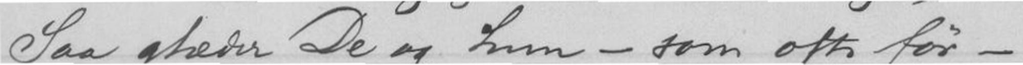Saa glæder De og hun - som ofte før -
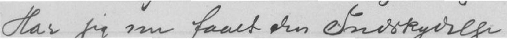Har jeg nu faaet den Indskydelse
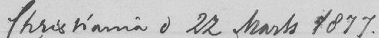Christiania d. 22 Marts 1877.
